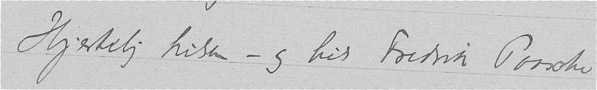Hjertelig hilsen – og hils Fredrik Paasche
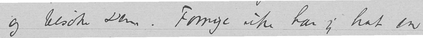og besøke Dem. Forrige uke har jeg hat en
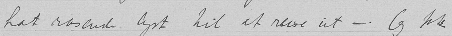hat rasende lyst til at reise ut – Og tak
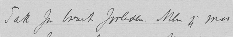Tak for brevet forleden. Men jeg maa
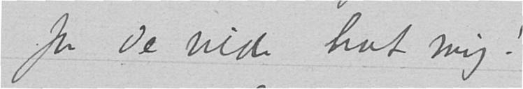for De vilde hat mig!
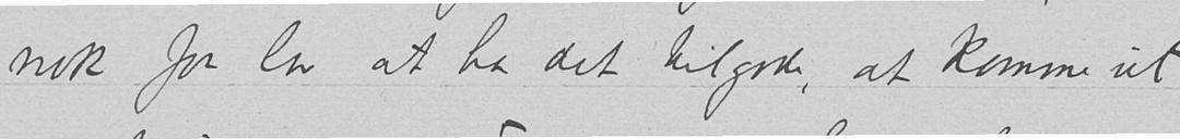nok faa lov at ha det tilgode, at komme ut
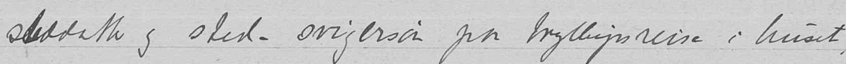steddatter og sted-svigersøn paa bryllupsreise i huset,
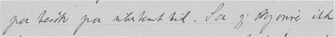paa besøk paa ubestemt tid. Saa jeg skjønner ikke
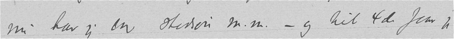nu har jeg en stedsøn m.m.– og til 4de faar jeg
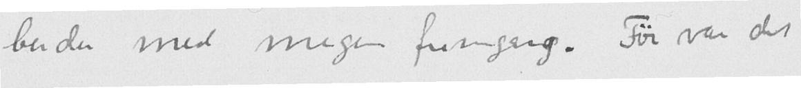beider med megen fremgang. Før var det
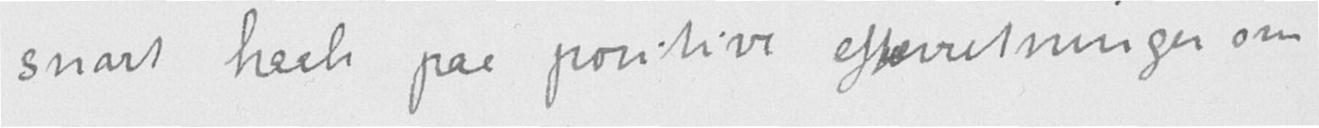snart haabe paa positive efterretninger om
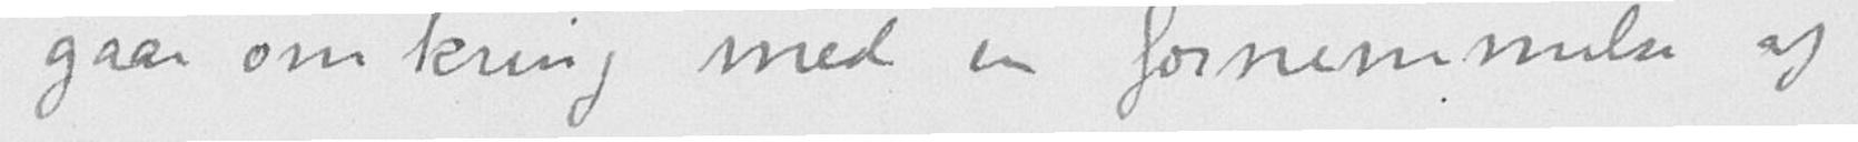gaar omkring med en fornemmelse af
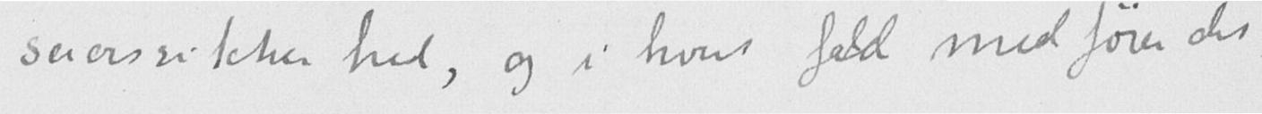seierssikkerhed, og i hvert fald medfører det
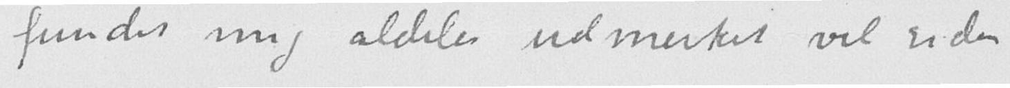fundet mig aldeles udmerket vel siden
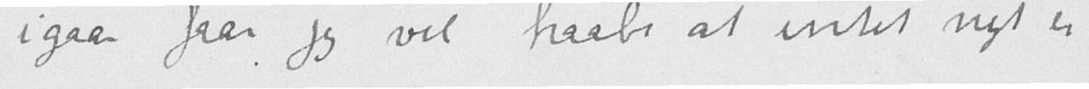igaar faar jeg vel haabe at intet nyt er
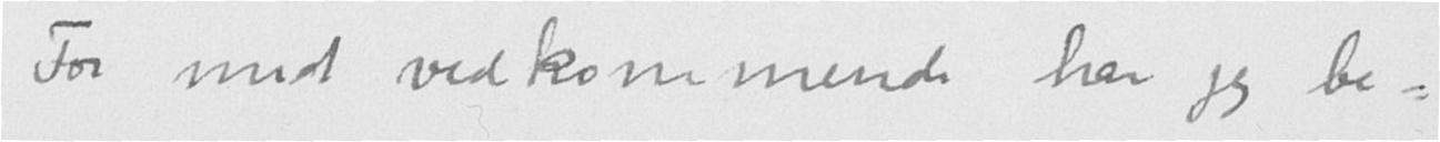For mit vedkommende har jeg be-
